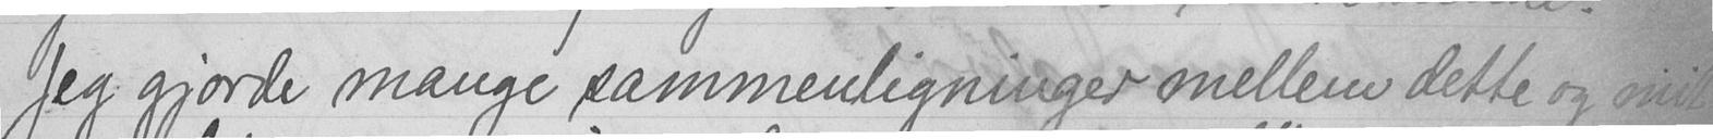Jeg gjorde mange sammenligninger mellem dette og mit
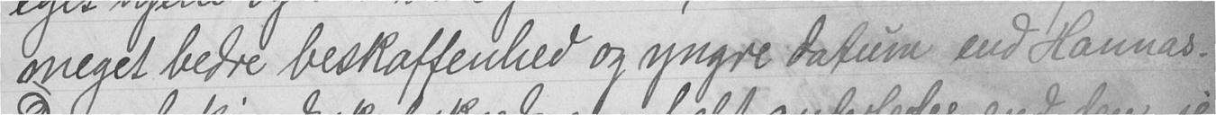meget bedre beskaffenhed og yngre datum end Hannas.
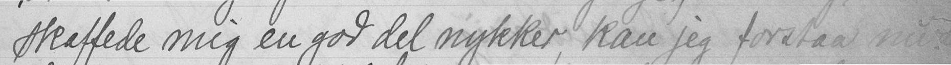skaffede mig en god del nykker kan jeg forstaa nu.
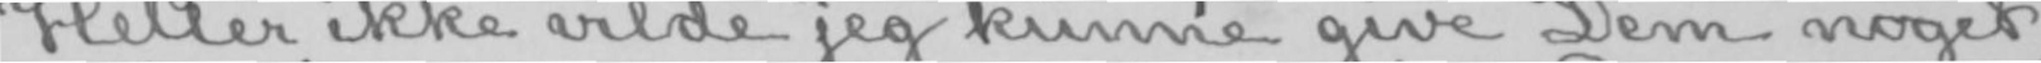Heller ikke vilde jeg kunne give Dem noget
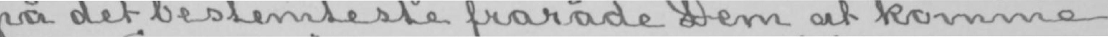på det bestemteste fraråde Dem at komme
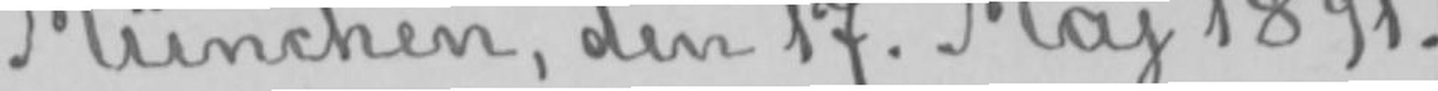München, den 17. Maj 1891.
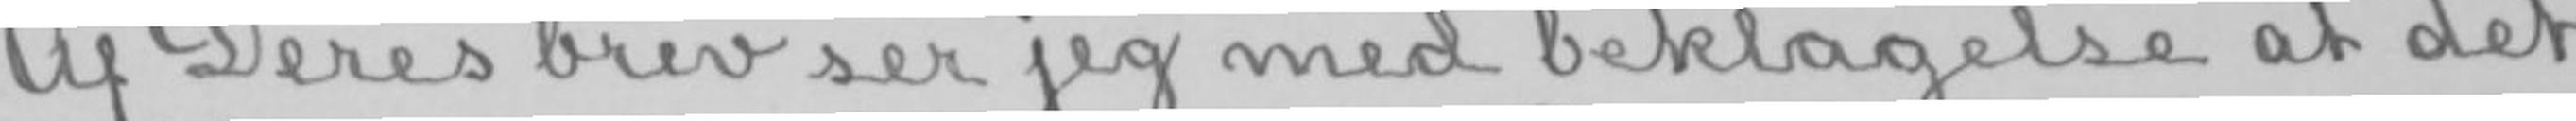Af Deres brev ser jeg med beklagelse at det
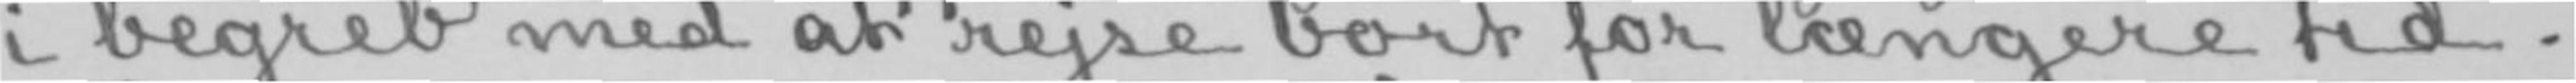i begreb med at rejse bort for længere tid.
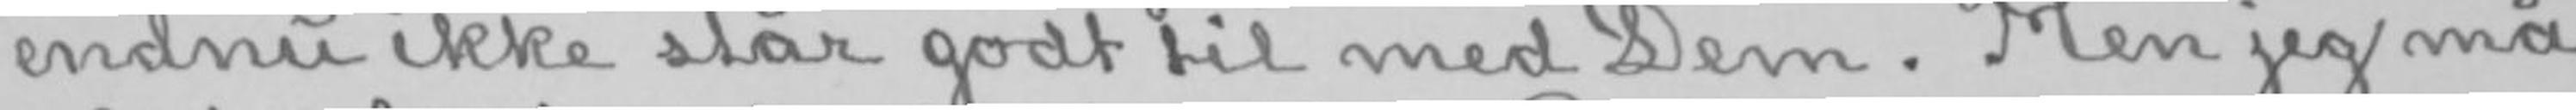endnu ikke står godt til med Dem. Men jeg må
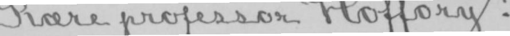Kære professor Hoffory!
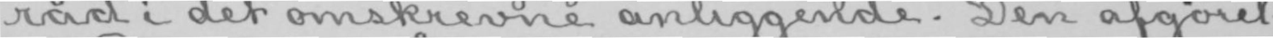råd i det omskrevne anliggende. Den afgørel-
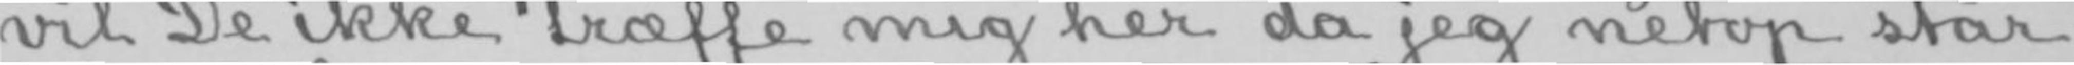vil De ikke træffe mig her da jeg netop står
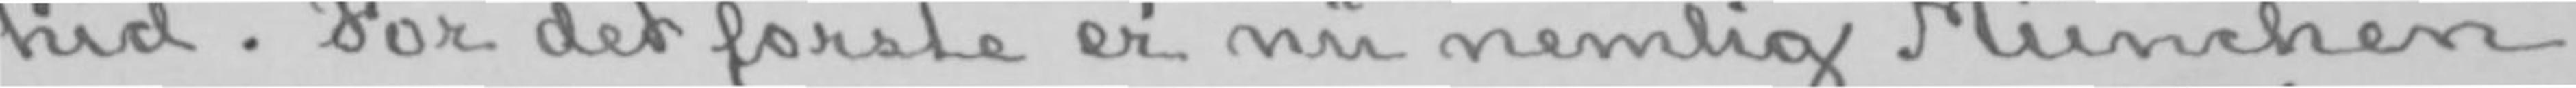hid. For det første er nu nemlig München
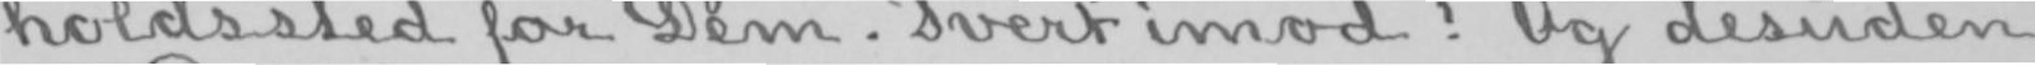holdssted for Dem. Tvert imod! Og desuden
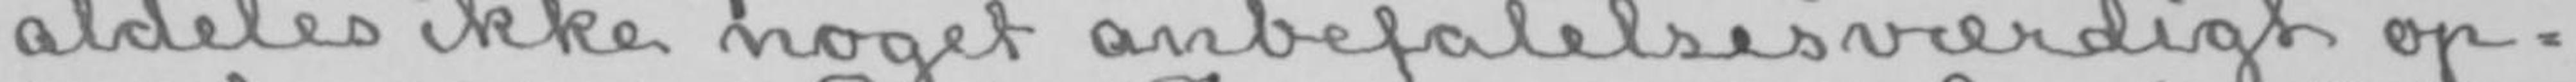aldeles ikke noget anbefalelsesværdigt op-
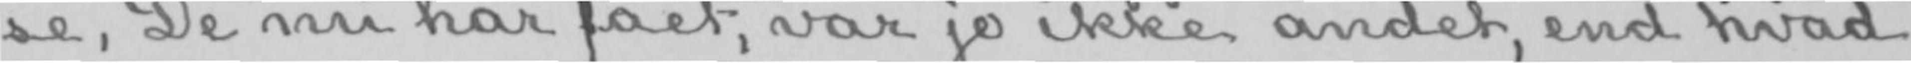se, De nu har fået, var jo ikke andet, end hvad
